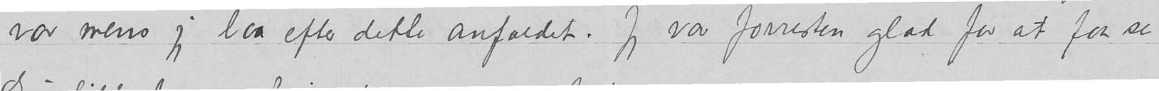var mens jeg laa efter dette anfaldet. Jeg var forresten glad for at faa se
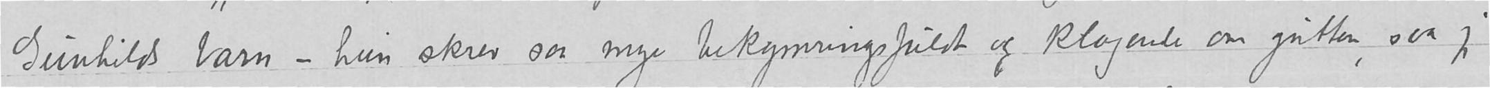Gunhilds barn – hun skrev saa mye bekymringsfuldt og klagende om gutten, saa jeg
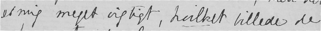er mig meget vigtigt, hvilket billede de
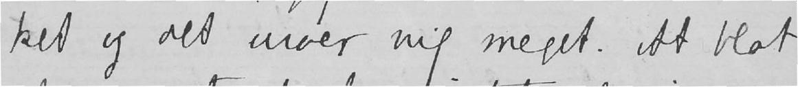ket og det uroer mig meget. At blot
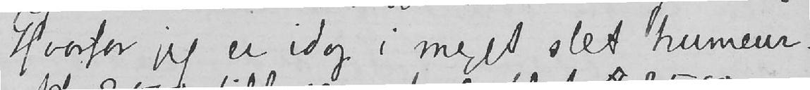Hvorfor jeg er idag i meget slet humeur.
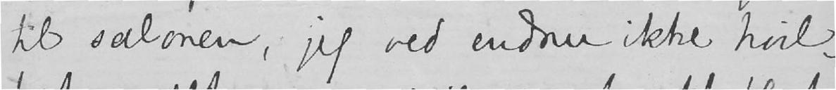til salonen, jeg ved endnu ikke hvil-
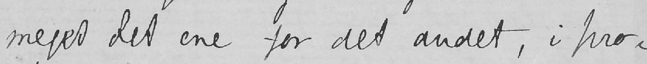meget det ene for det andet, i pro-
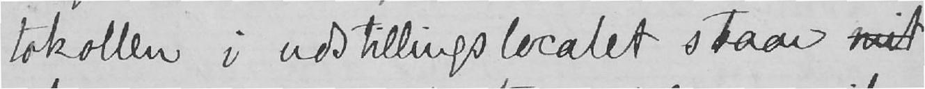tokollen i udstillingslocalet staar mit
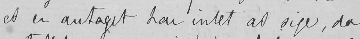et er antaget har intet at sige, da
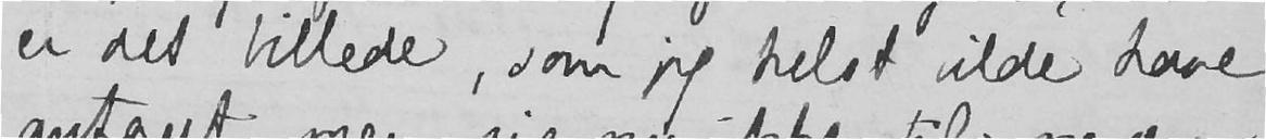er det billede, som jeg helst vide have
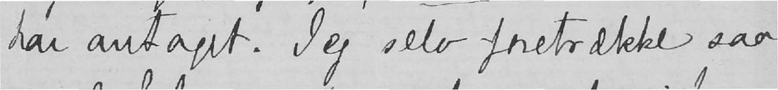har antaget. Jeg selv foretrække saa
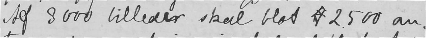Af 8000 billeder skal blot 4 2500 an-
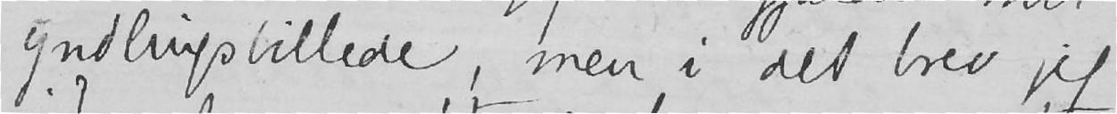yndlingsbillede, men i det brev jeg
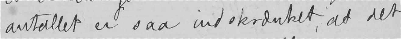antallet er saa indskrænket, at det
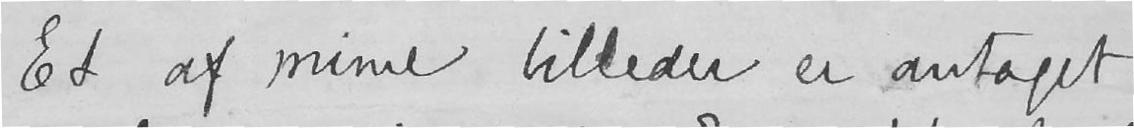Et af mine billeder er antaget
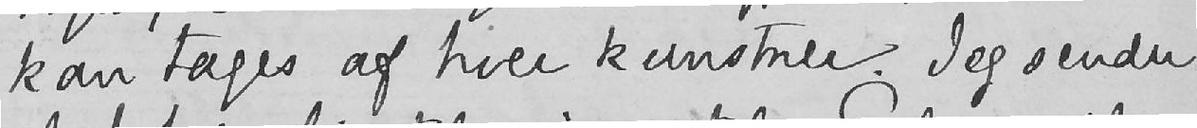kan tages af hver kunstner. Jeg sender
